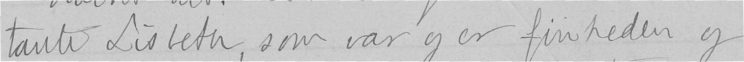tante Lisbeth, som var og er finheden og
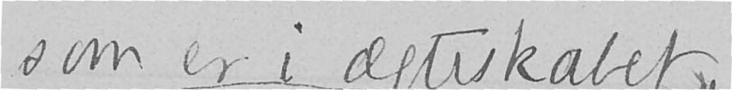som er i ægteskabet,
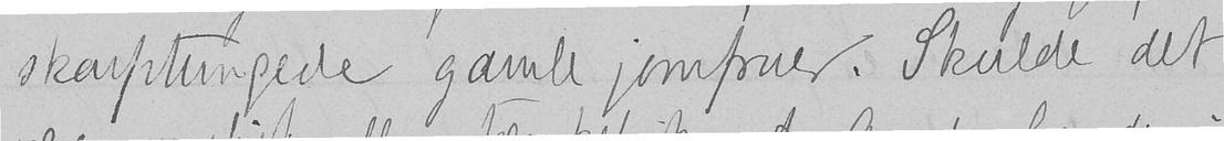skarptungede gamle jomfruer. Skulde det
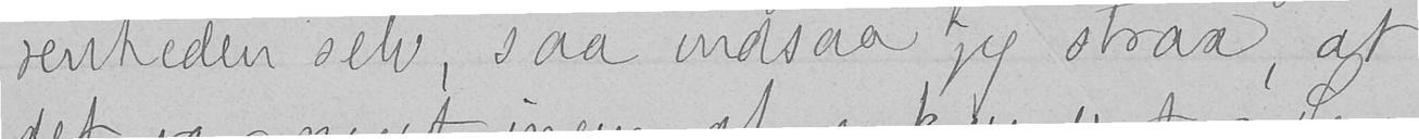renheden selv, saa indsaa jeg strax, at
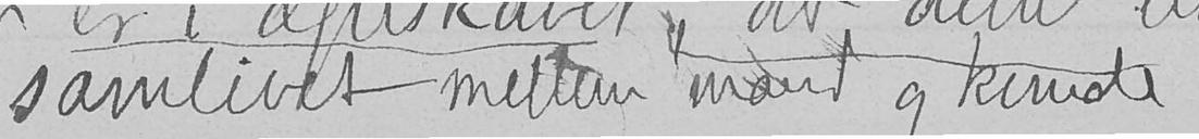samlivet mellem mand og kvinde
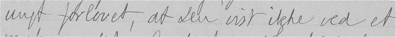ungt forlovet, at Du vist ikke ved et
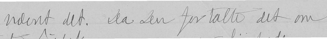nævnt det. Da Du fortalte det om
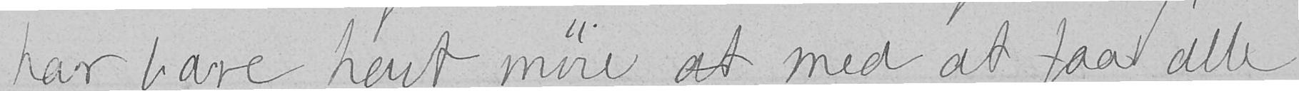har bare havt møie at med at faa alle
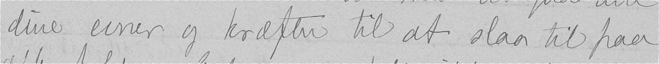dine evner og kræfter til at slaa til paa
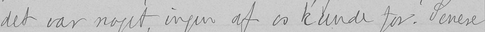det var noget ingen af os kunde for. Senere
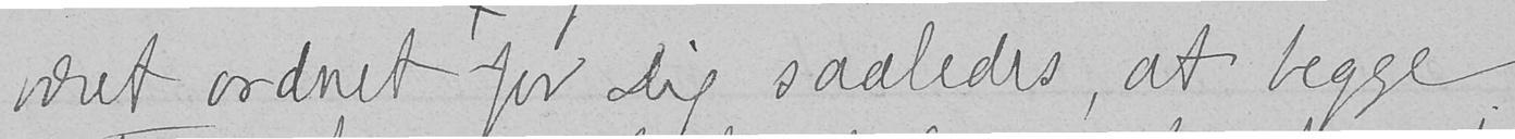været ordnet for Dig saaledes, at begge
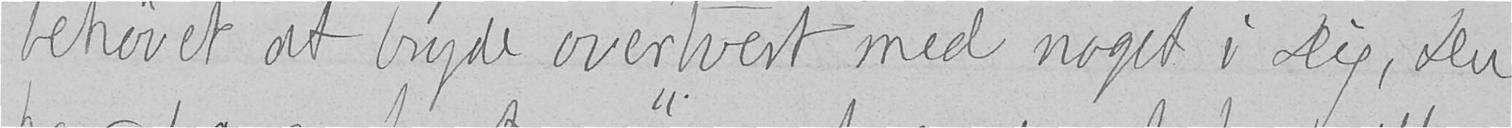behøvet at bryde over tvert med noget i Dig, Du
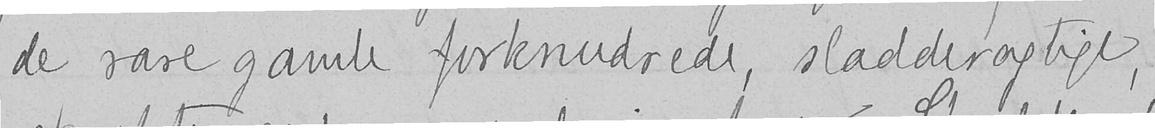de rare gamle forknudrede, sladderagtige,
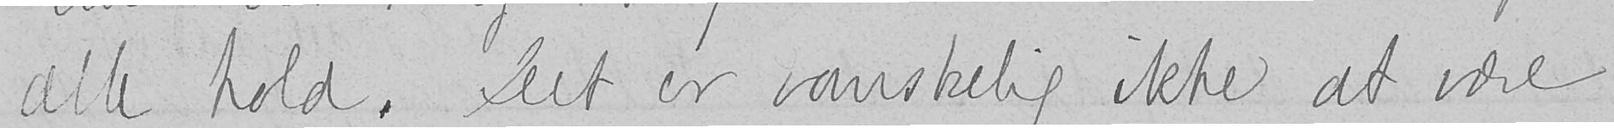alle hold. Det er vanskelig ikke at være
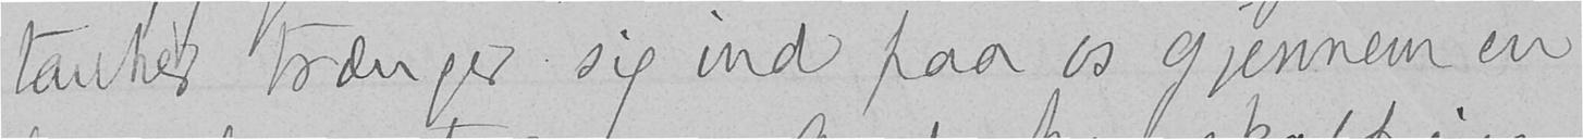tanker trænger sig ind paa os gjennem en
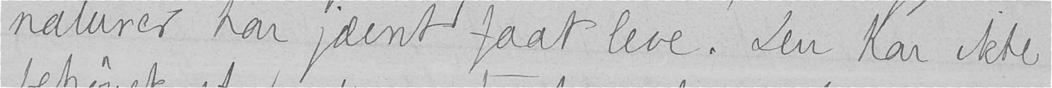naturer har jævnt faat leve. Du har ikke
